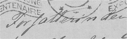Forfatterinden
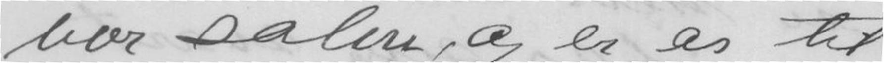vor salon, og er os til
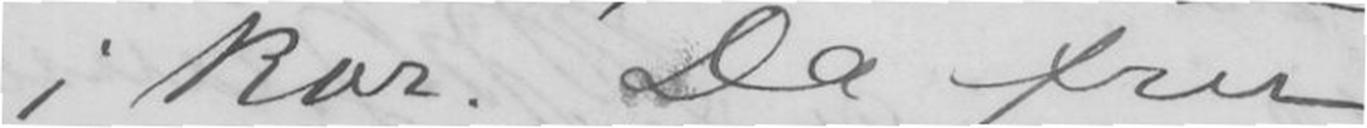i kor. Da fru
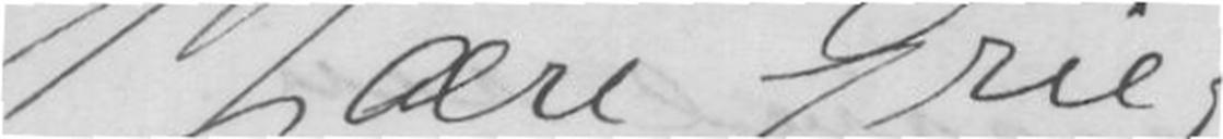Kjære Grieg
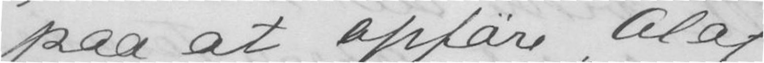paa at opføre Olaf
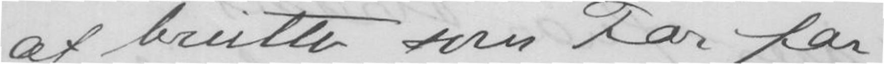af brutto som Far for
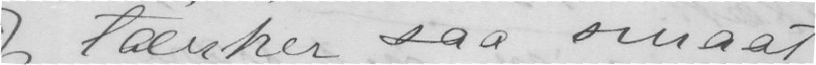Jeg tænker saa smaat
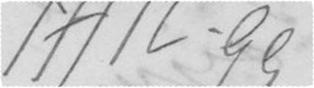17/12-99
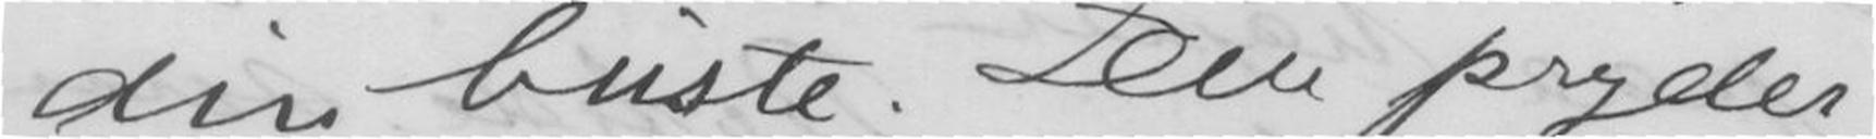din byste. Den pryder
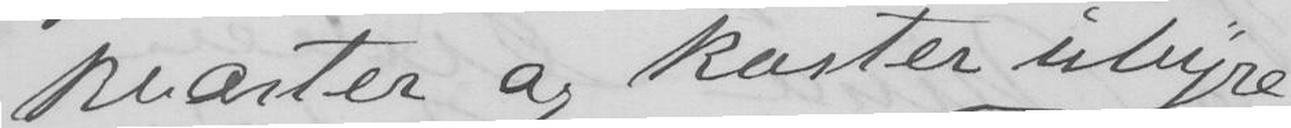kvarter og koster uhyre
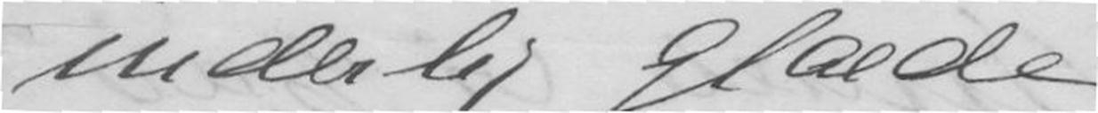inderlig glæde.
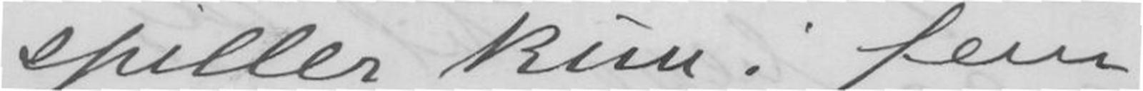spilles kun i fem
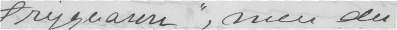Trygvason, men du
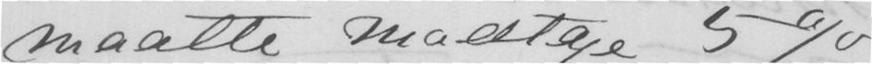maatte modtage 5%
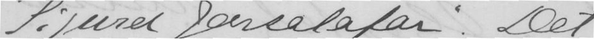Sigurd Jordsalafar. Det
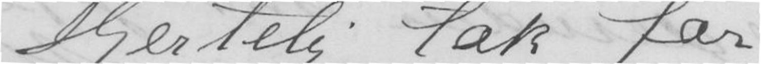Hjertelig tak for
